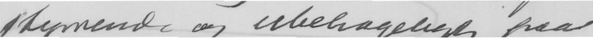styrrende og ubehageligt paa
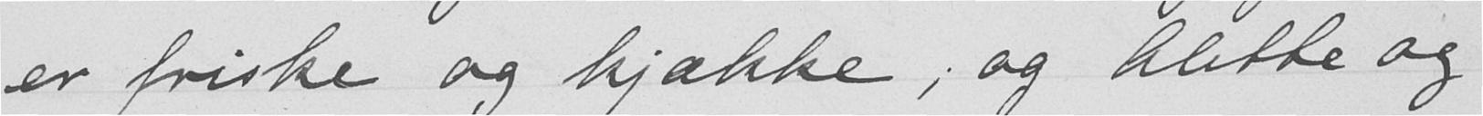er friske og kjække, og Alette og
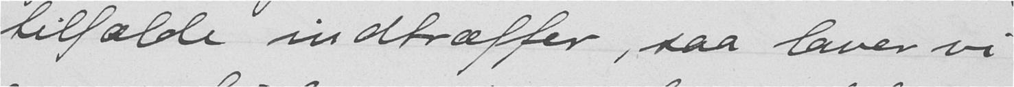tilfælde indtræffer, saa laver vi
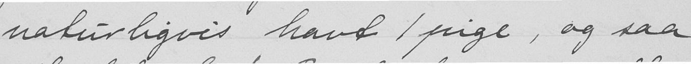naturligvis havt 1 pige, og saa
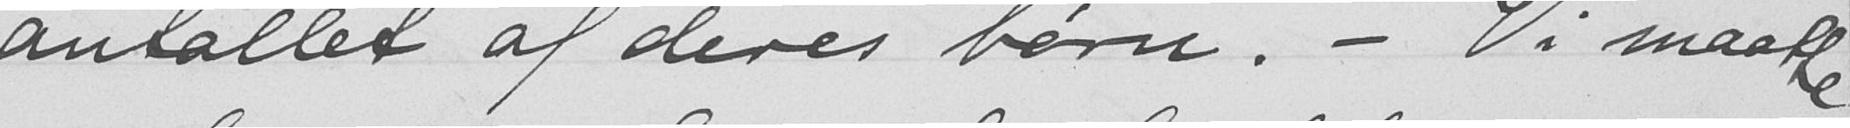antallet af deres børn. - Vi maatte
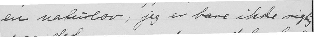en naturlov; jeg er bare ikke rigtig
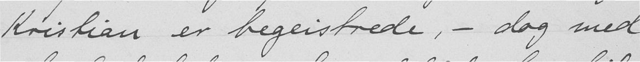Kristian er begeistrede, - dog med
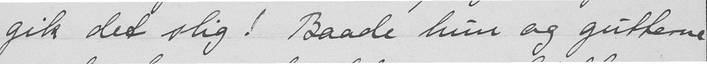gik det slig! Baade hun og gutterne
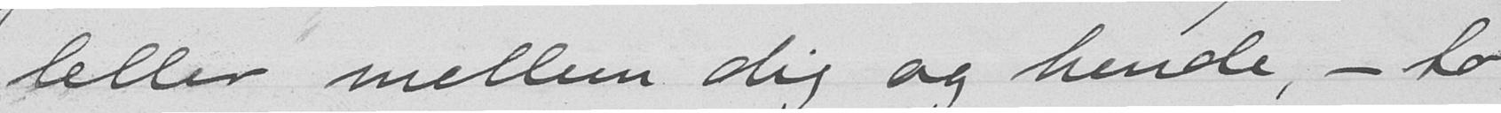leller mellem dig og hende, - to
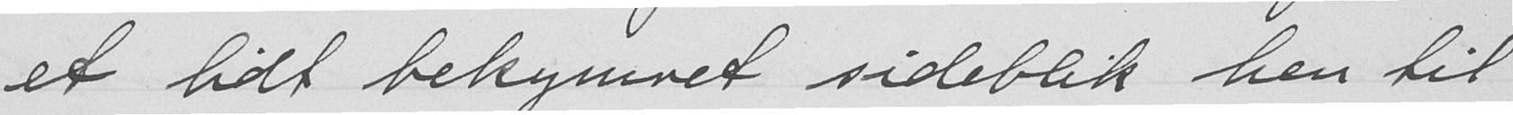et lidt bekymret sideblik hen til
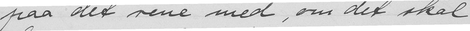paa det rene med, om det skal
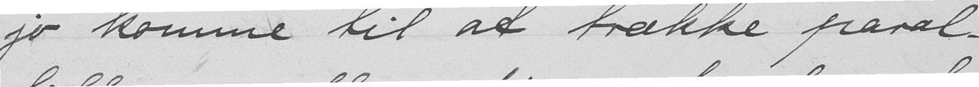jo komme til at trække paral-
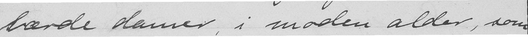lærde damer, i moden alder, som
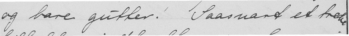og bare gutter! Saasnart et tredie
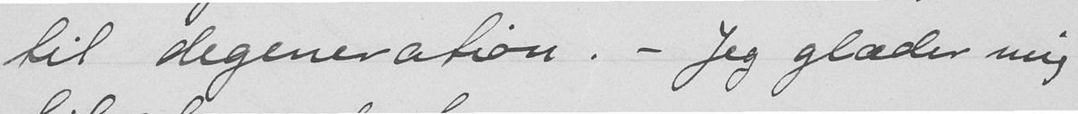til degeneration. - Jeg glæder mig
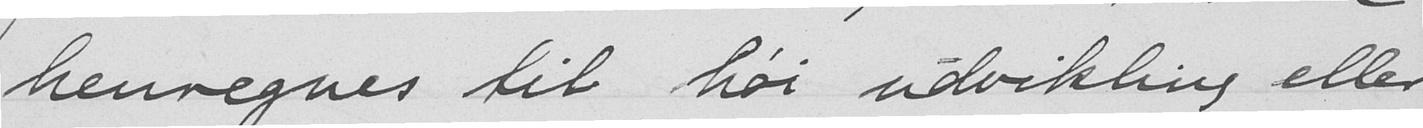henregnes til høi udvikling eller
